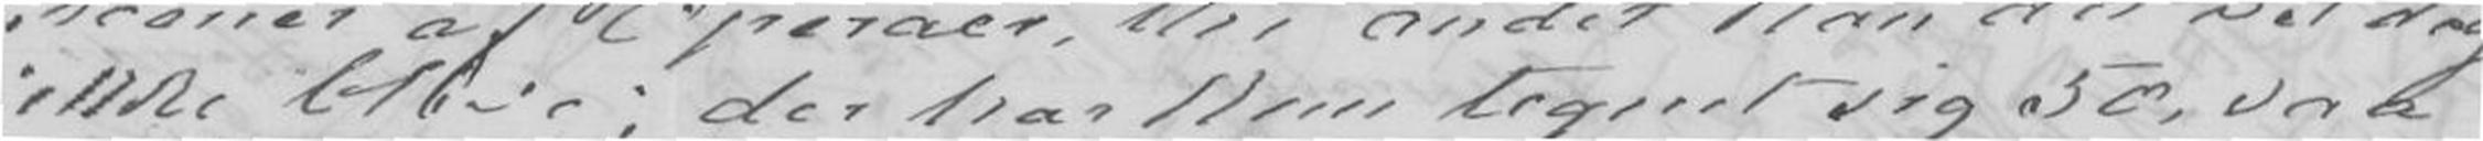ikke blive; der har kun tegnet sig 50, saa
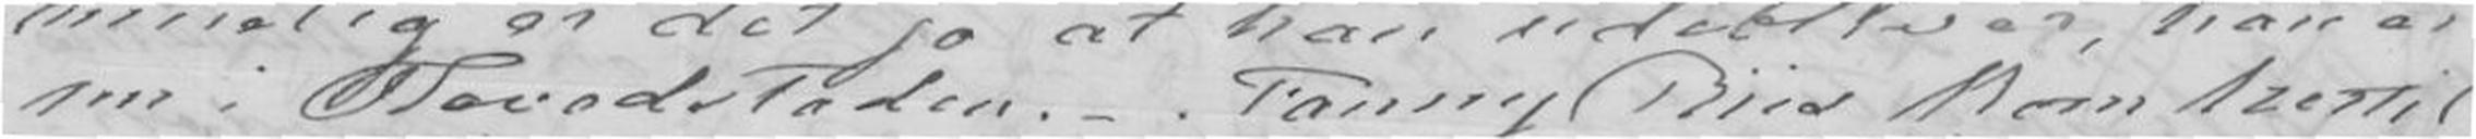nu i Hovedstaden. - Fanny Riis kom hertil
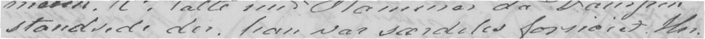standsede der, han var særdeles fornøiet. Hu-
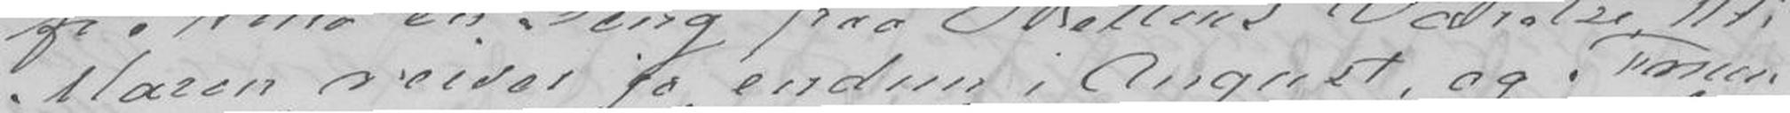Maren reiser jo endnu i August, og Fruen
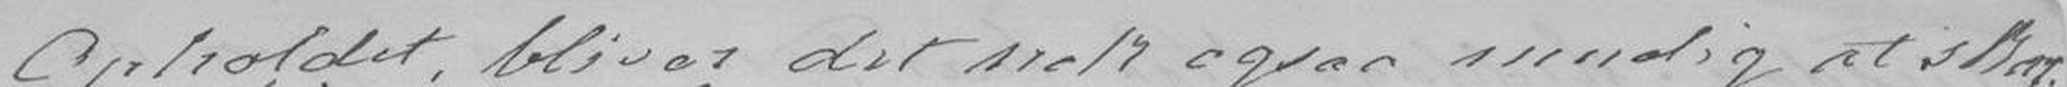Opholdet bliver det nok ogsaa mulig at skaf-
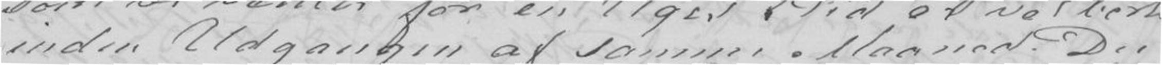inden Udgangen af samme Maaned. Du
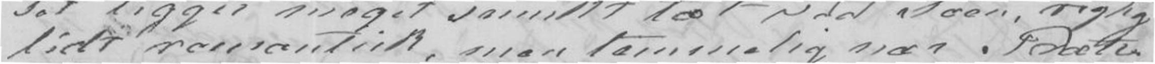lidt romantisk, men temmelig nær Præste-
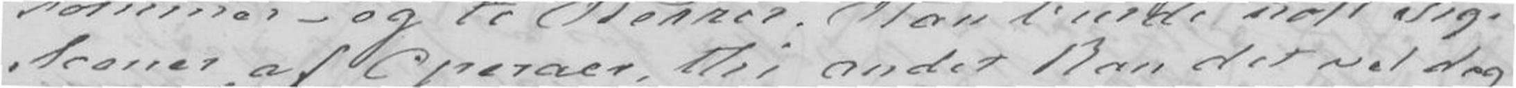Scener af Operaen, thi andet kan det vel dog
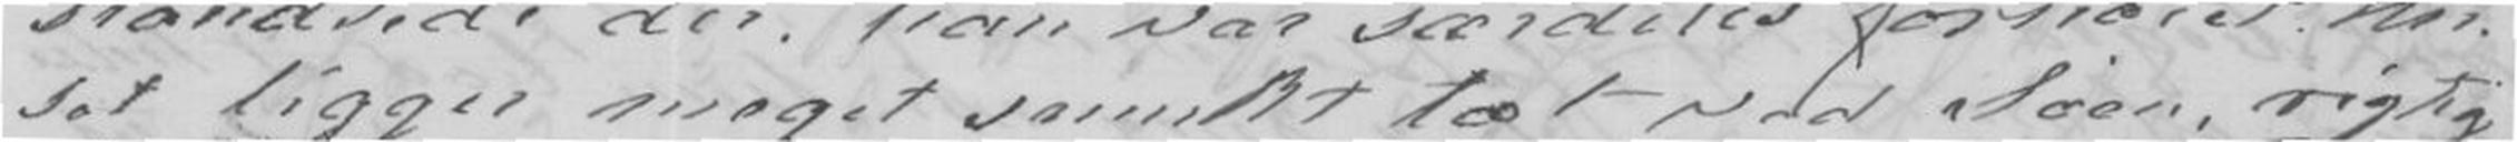set ligger meget smukt tæt ved Søen, rigtig
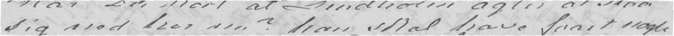sig ned her nu? han skal have faaet nogle
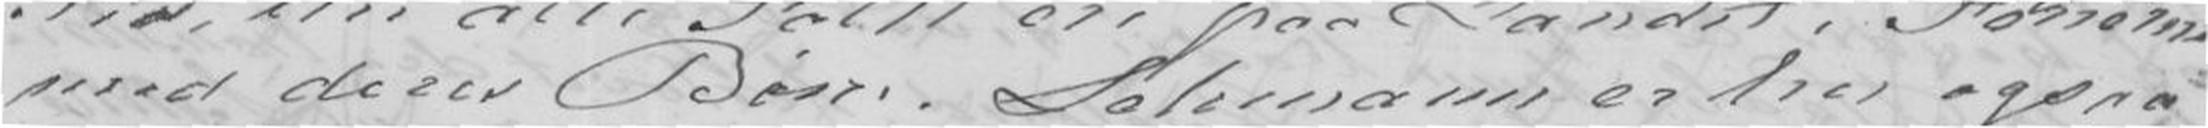med deres Børn. Lohmann er her ogsaa
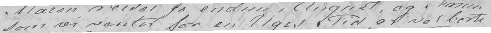som vi venter for en Uges Tid er vel borte
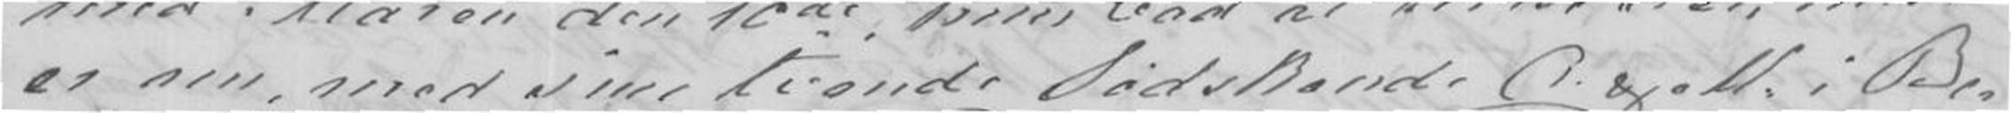er nu med sine tiende Sødskende O. og M. i Be-
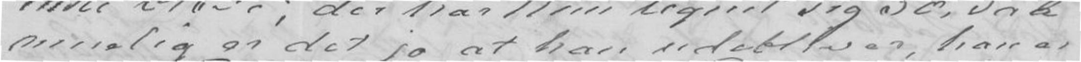muelig er det jo at han udebliver, han er
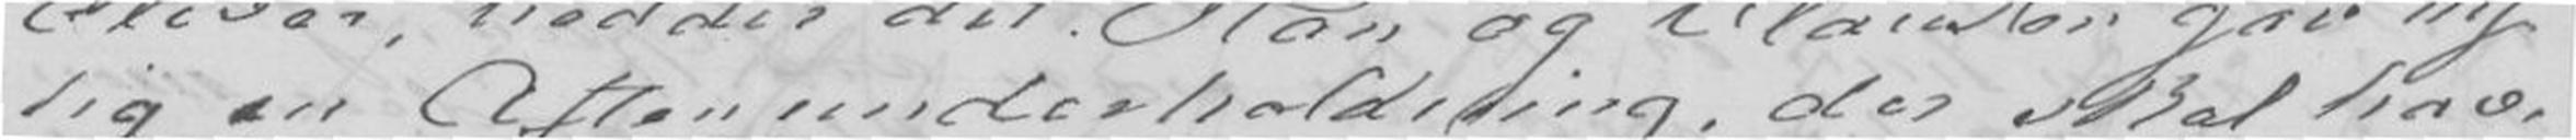lig en Aftenunderholdning, der skal have
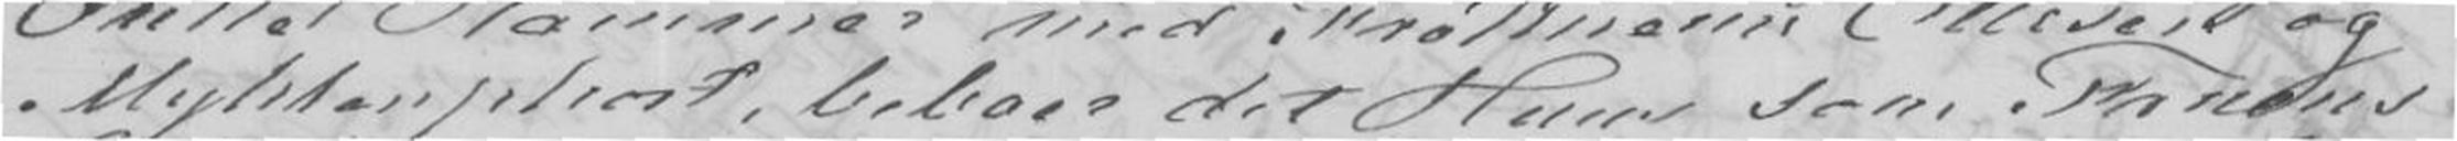Myhlenphost, beboer det Huus som Fruens
